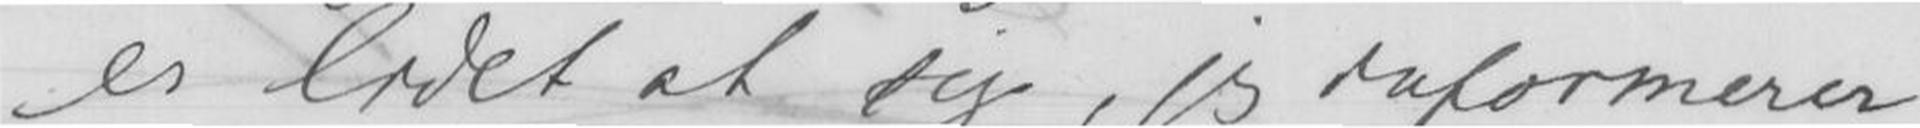er lidet at sige, jeg indformerer
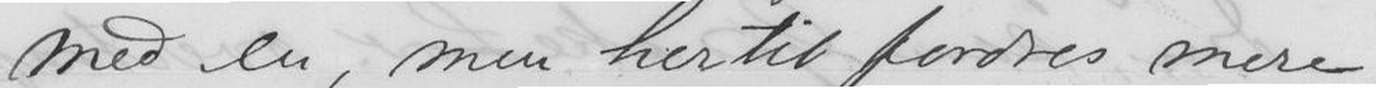med en, men hertil fordres mere
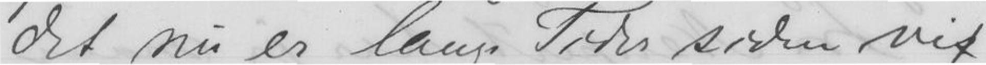det nu er lange Tider siden vi
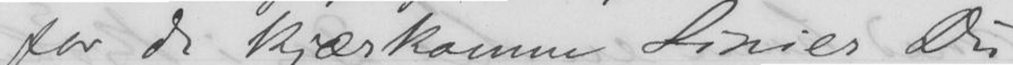Brev for de kjærkomne Linier Du
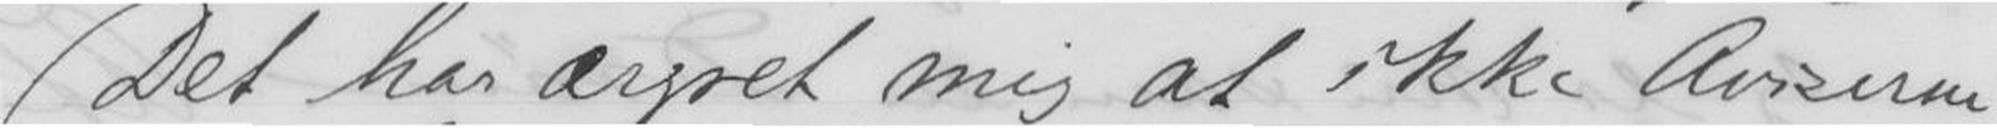Det har ærgret mig at ikke Aviserne
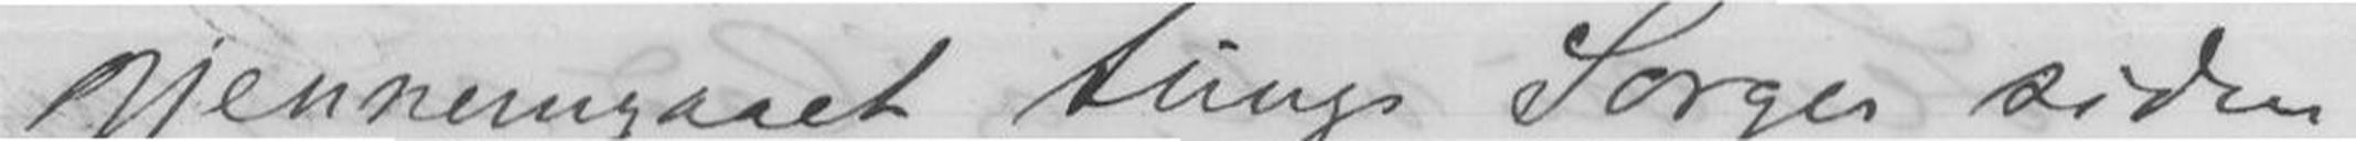gjennemgaaet tungs Sorger siden
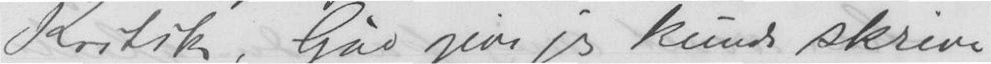Kritik. Gud give jeg kunde skrive
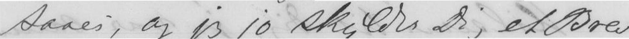saaes, og jeg jo skylder Dig et
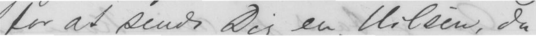for at sende Dig en Hilsen, da
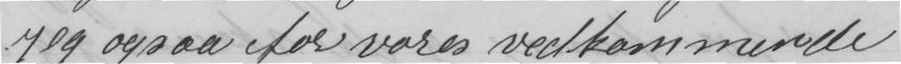jeg ogsaa for vores vedkommende
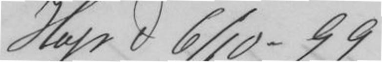Hop d 6/10 - 99
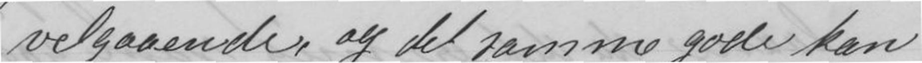velgaaende, og det samme gode kan
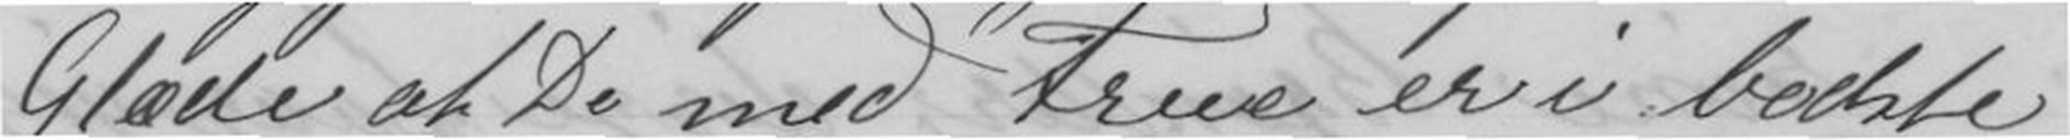Glæde at De med Frue er i bedste
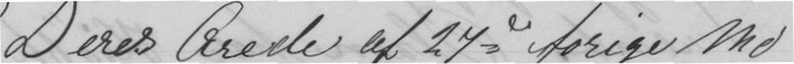Deres Ærede af 27de forige Md
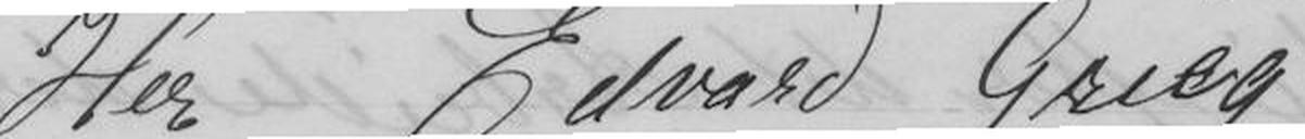Herr Edvard Grieg
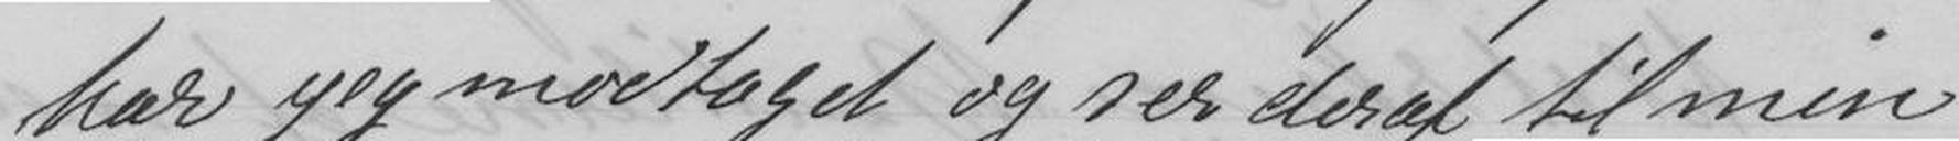har jeg modtaget og ser deraf til min
